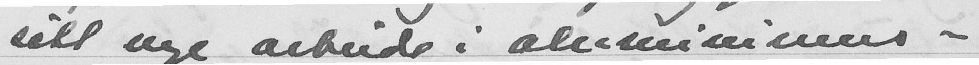sitt nye arbeide i aliminiums-
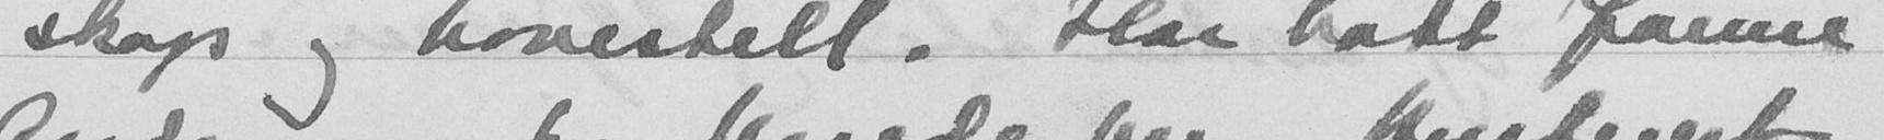skap og hovestelt. Har hatt Janne
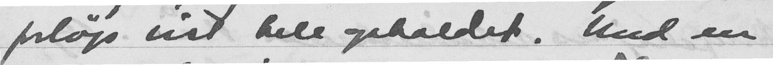forløp vist hele opholdet. Med en
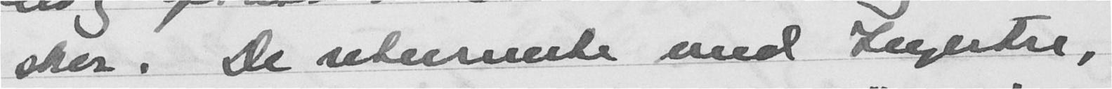sker. De setusmte med Kyeitse,
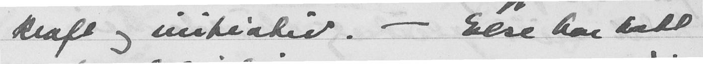kraft og intiativ. - Else har hatt
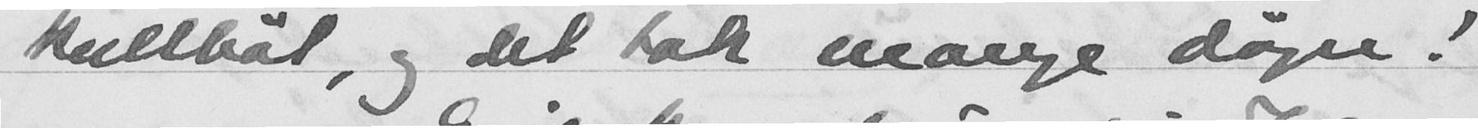kullbåt, og det tok mange døgn!
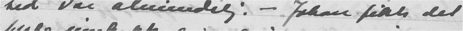tid var almindelig. - Johan fikk det
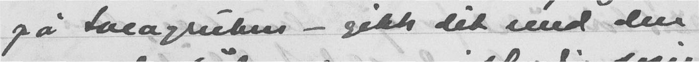på Sveagruben - gikk dit med den
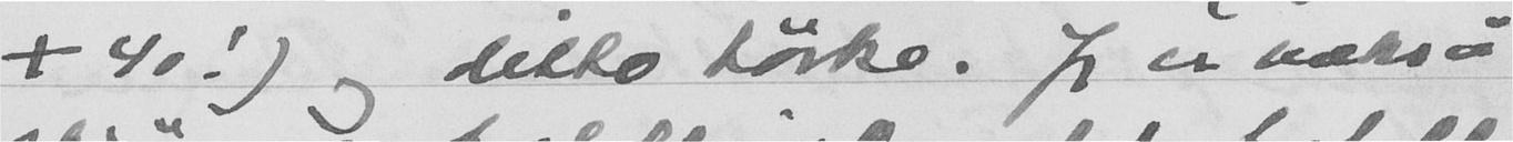+ 40!) og ditto tørke. Jeg er nokså
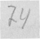74
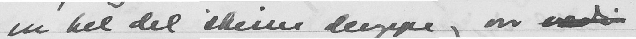en hel del skisser deroppe og var
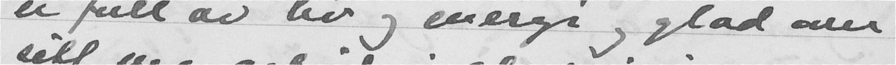er full av liv og energi og glad om
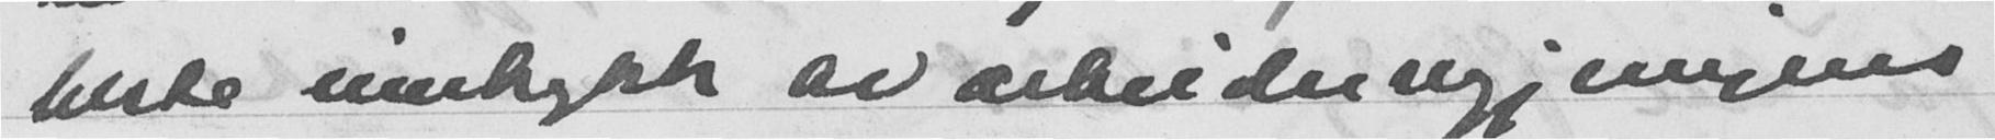beste inntrykk av arbeiderregjeringens
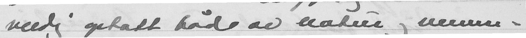veldig optatt både av natur og menne-
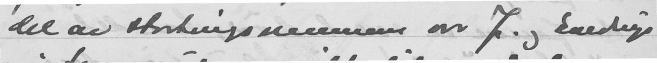del av stortingsmennene var J. og Sverdrups
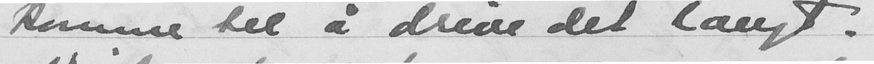komme til å drive det langt.
